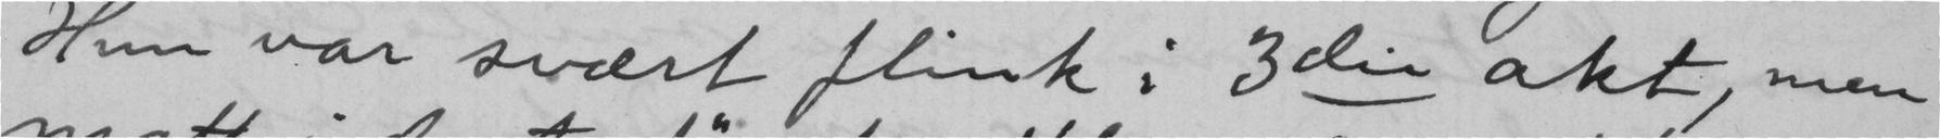Hun var svært flink i 3die akt, men
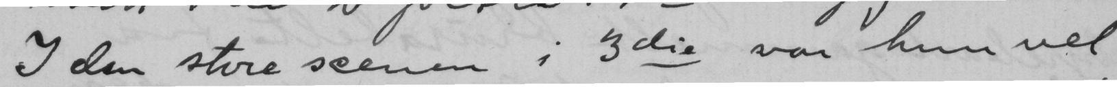I den store scenen i 3die var hun vel
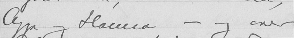Agga og Hanna - og over
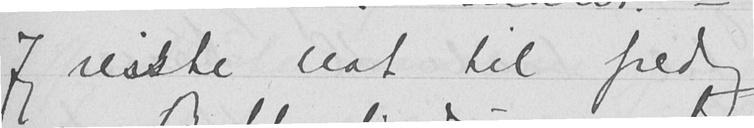Jeg reiste nat til fredag
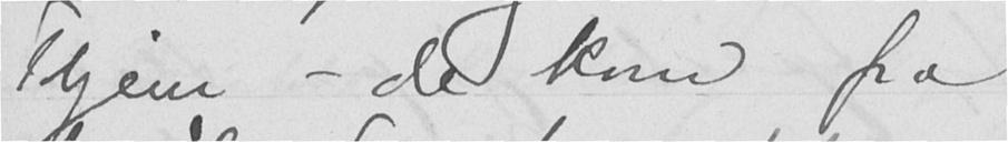Thjem - de kom fra
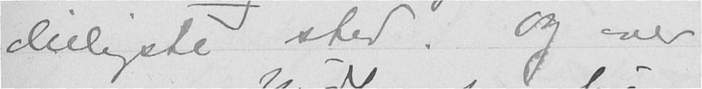deiligste sted. Og over
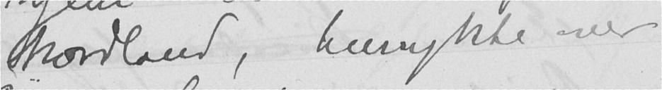Nordland, henrykte over
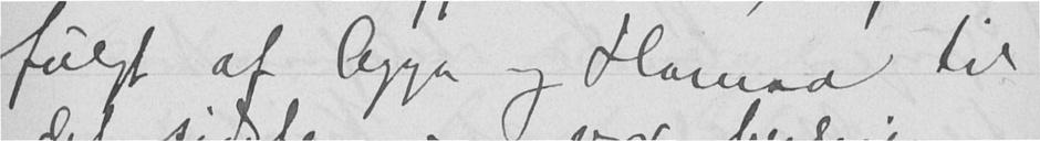fulgt af Agga og Hanna til
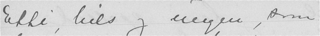Etti, Nils og ungen, som
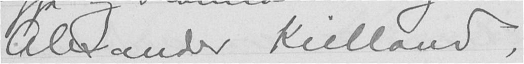Alexander Kielland,
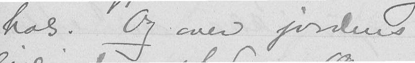hos. Og over jordens
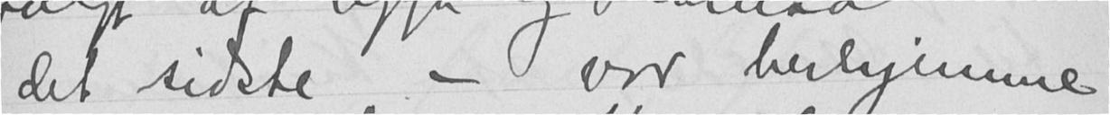det sidste -  var herhjemme
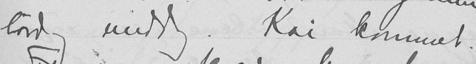lørdag middag. Kai kommet.
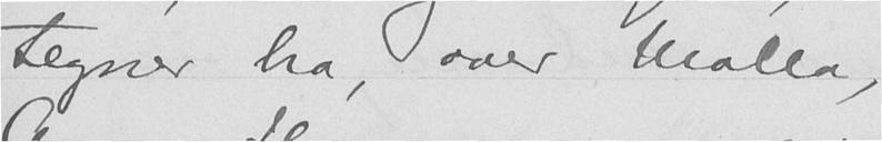tegner bra over Malla,
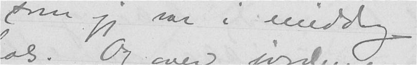som jeg var i middag
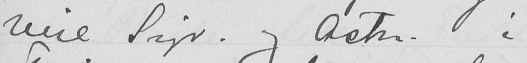veie Sigr. og Astr. i
